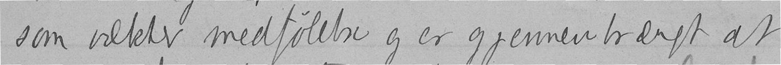som vækker medfølelse og er gjennemtrængt af
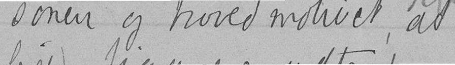sonen og hovedmotivet, at
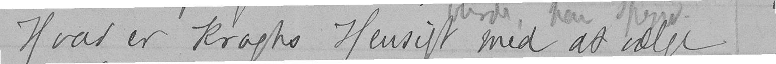Hvad er Kroghs Hensigt med at vælge
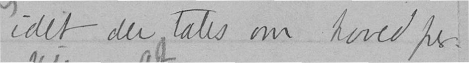idet der tales om hoved per-
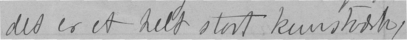det er et helt stort kunstværk,
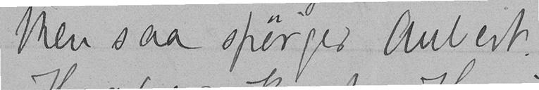Men saa spørger Aubert.
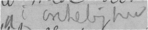"i virkelighed
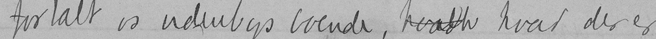fortalt os udenbys boende, hvadh hvad der er
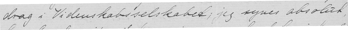drag i Videnskabsselskabet; jeg synes absolut,
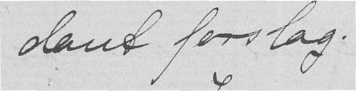dant forslag.
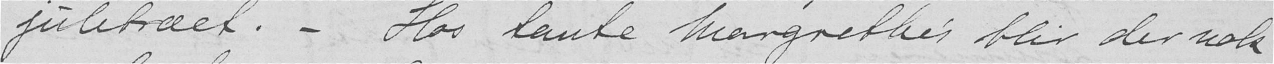juletræet. – Hos tante Margrethe's blir der nok
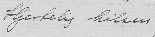Hjertelig hilsen
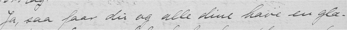Ja, saa faar du og alle dine have en glæ-
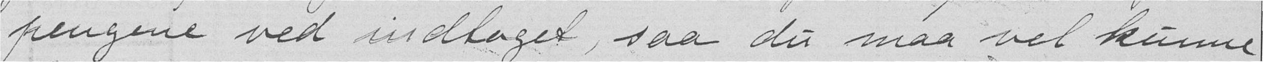pengene ved indtoget, saa du maa vel kunne
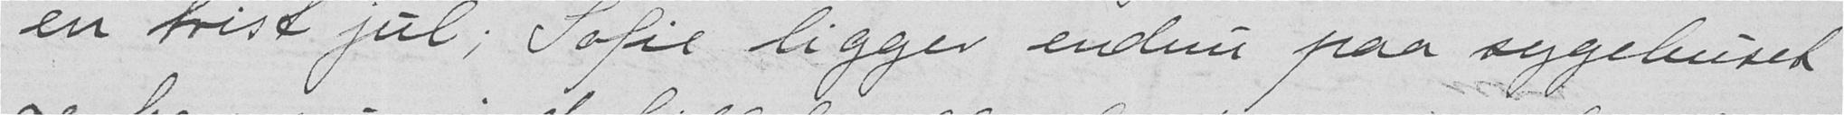en trist jul; Sofie ligger endnu paa sygehuset
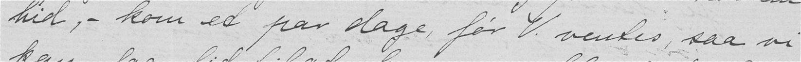hid, – kom et par dage, før V. ventes, saa vi
